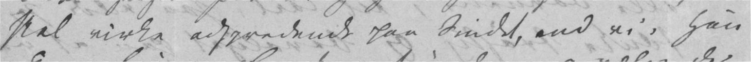skal virke adspredende paa Sindet, end vi, Han
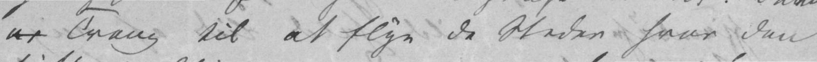er Trang til at flye de Steder hvor den
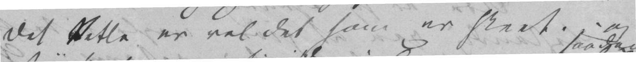Det Rette er vel det som er skeet, og
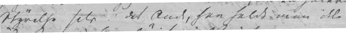Styrelse selv i det Onde, saa holdt man ikke
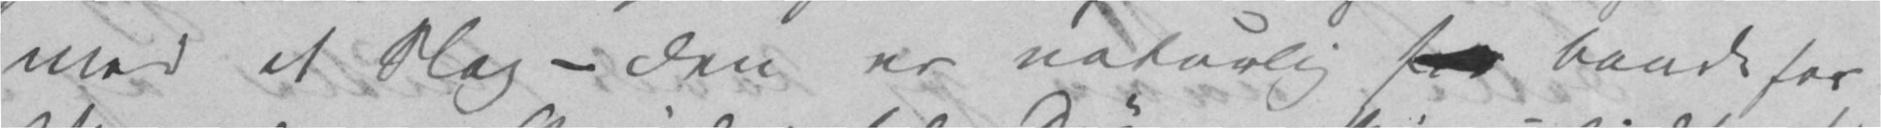med et Slag – den er naturlig for baade for
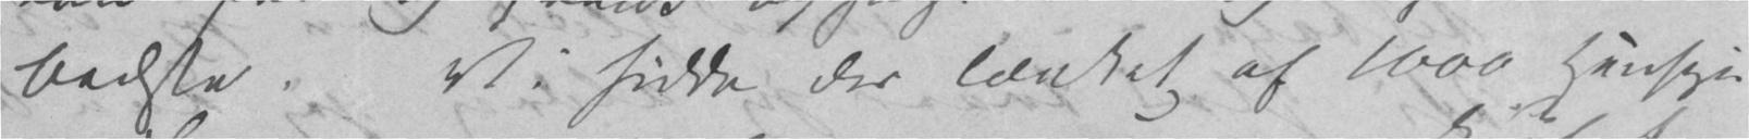bedste. Vi sidde der lænket af 1000 Hensyn
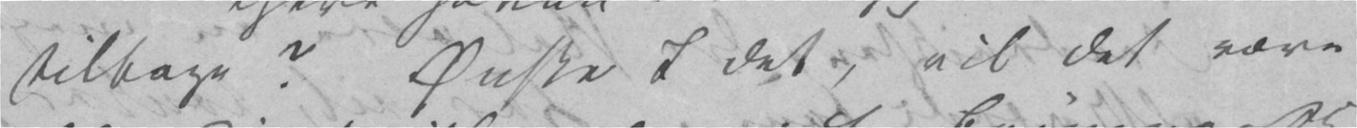tilbage? Ønske I det, vil det være
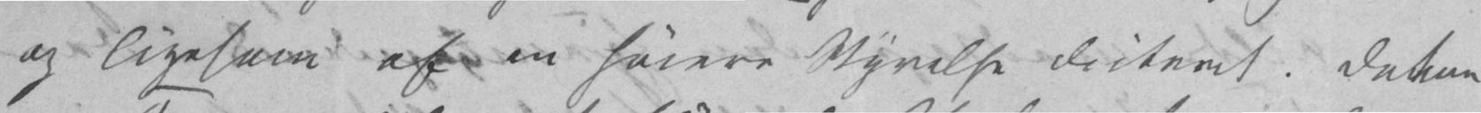og ligesom af en høiere Styrelse dicteret. Denne
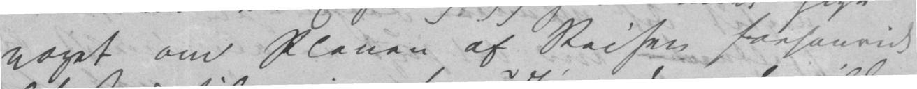noget om Planen af Reisen forsaavidt
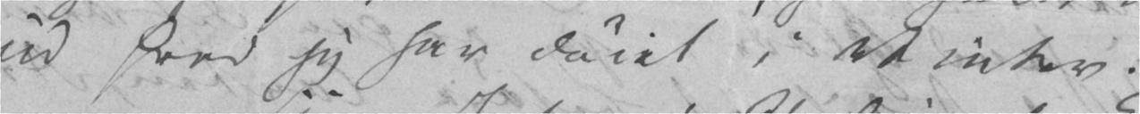ud hvad jeg har døiet i Vinter.
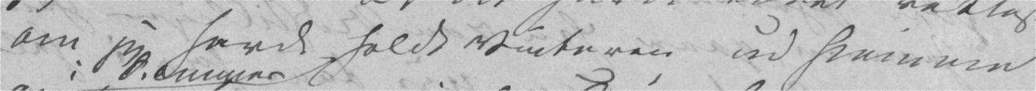om jeg havde holdt Vinteren ud hjemme
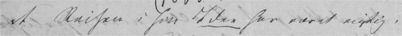at Reisen i sin Idee har været rigtig,
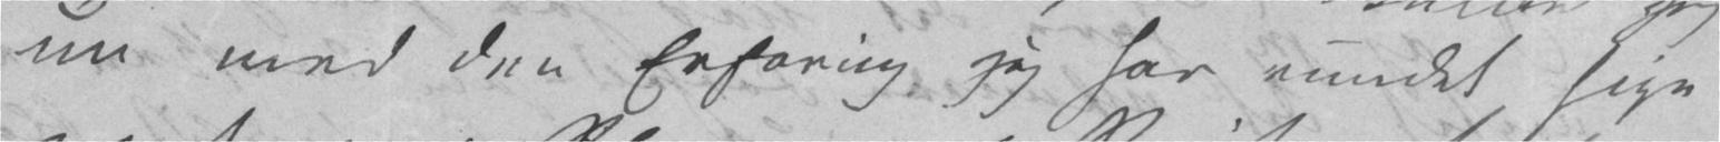nu med den Erfaring jeg har vundet sige
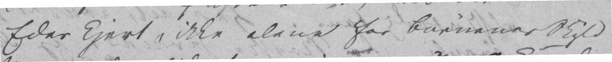Eder kjert, ikke alene for Børnenes Skyld
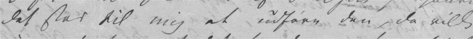det stod til mig at udføre den, da vilde
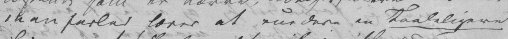man forlod lærer at vurdere en Taaleligere
